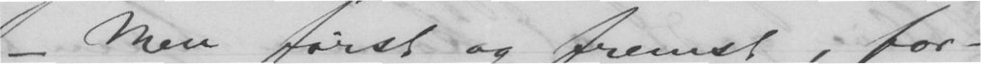- Men først og fremst; for-
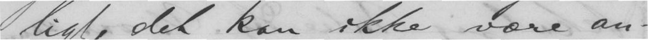ligt, det kan ikke være an-
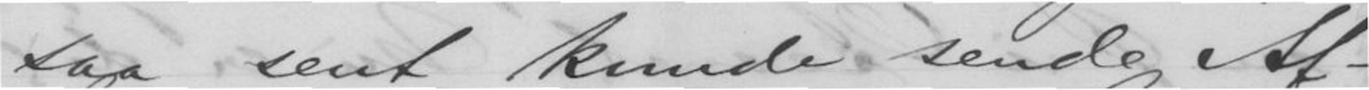saa sent kunde sende Af-
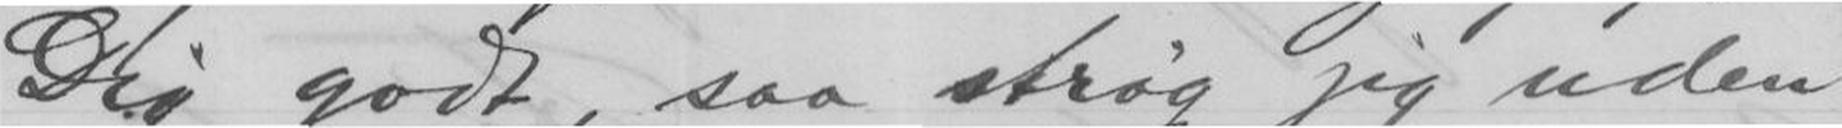Dig godt, saa strøg jeg uden
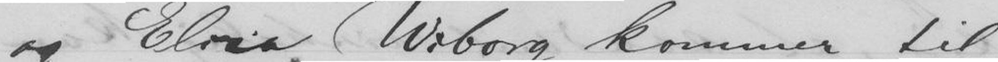og Elisa Wiborg kommer til
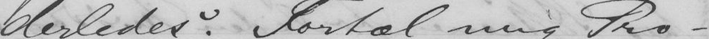derledes. Fortæl mig Pro-
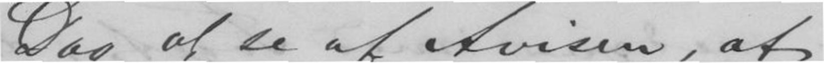Dag at se af Avisen, at
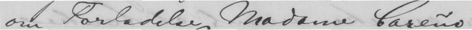om Forladelse Madam Careño
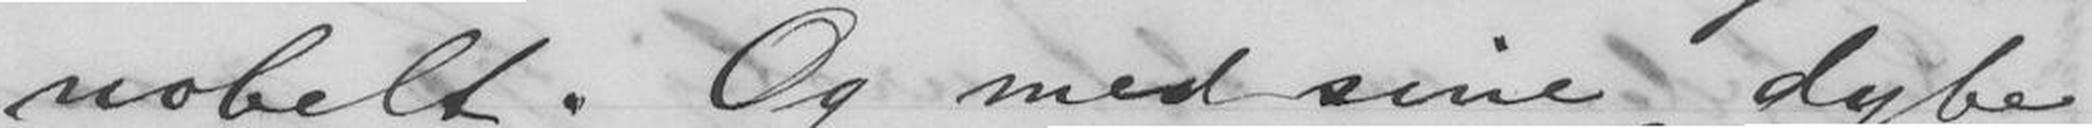nobelt. Og med sine dybe
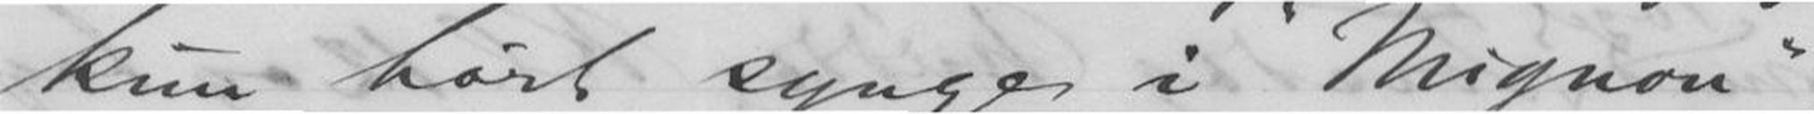kun hørt synge i "Mignon",
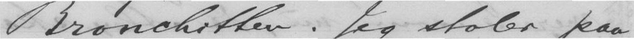Bronchitten. Jeg stoler paa
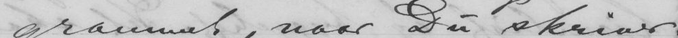grammet, naar Du skriver.
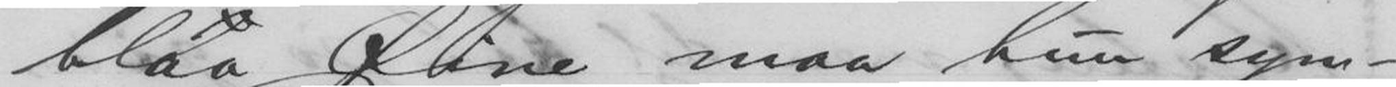blaa Øine maa hun syn-
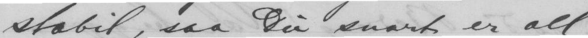stabil, saa Du snart er all
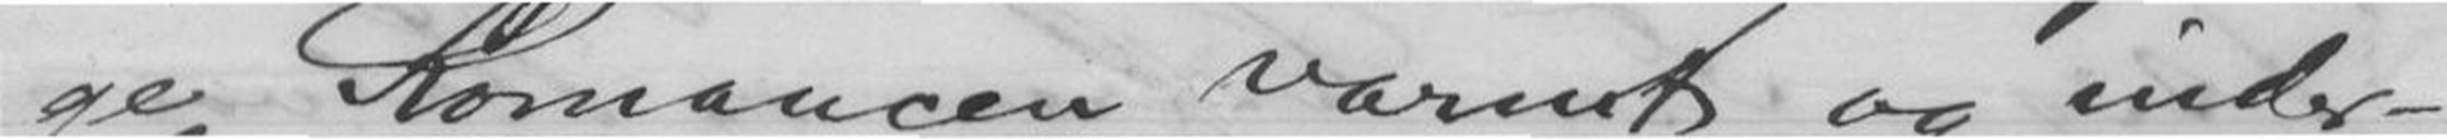ge Romancen varmt og inder-
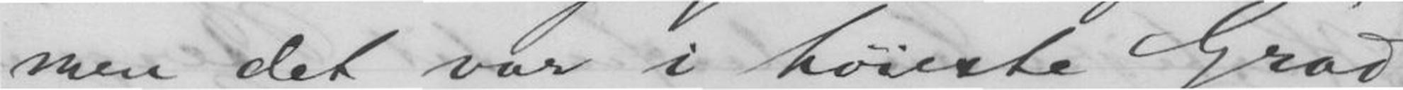men det var i Høieste Grad
